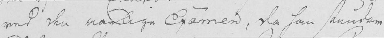ved den aarlige Examen, da han stundom
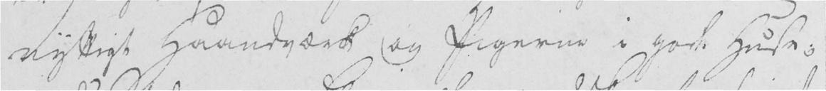nyttigt Haandværk og Pigerne i gode Huse;
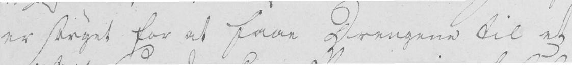er sørget for at faae Drengene til et
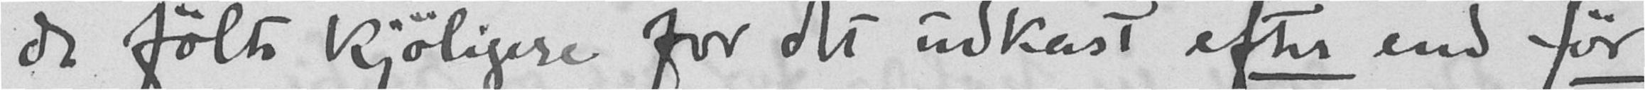de følte kjøligere for det udkast efter end før
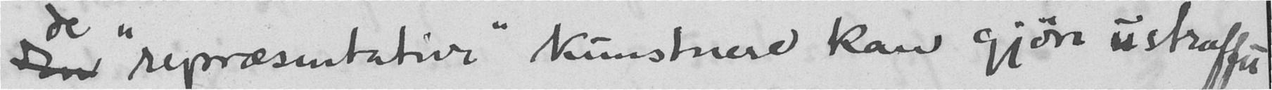Den "repræsentative" kunstnere kan gjøre ustraffet
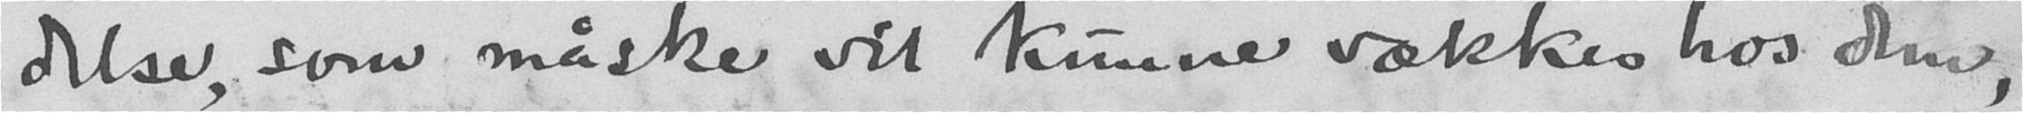delse, som måske vil kunne vækkes hos dem,
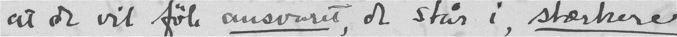at de vil føle ansvaret, de står i, stærkere
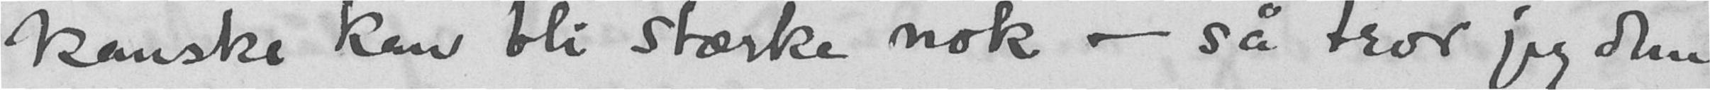kanske kan bli stærke nok - så tror jeg dem
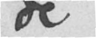De
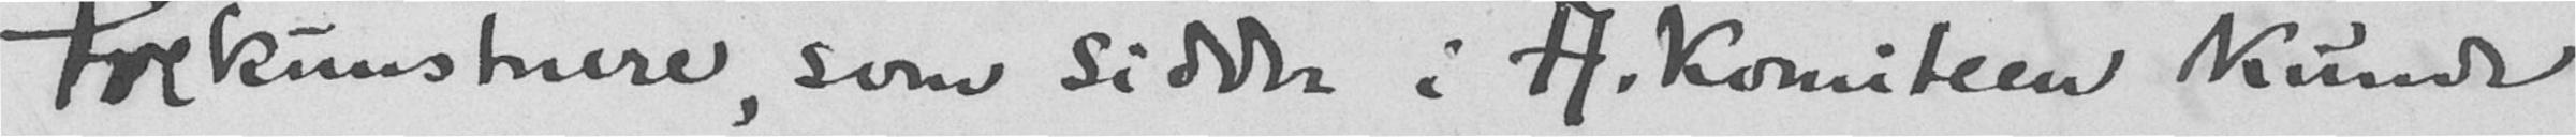tre kunstnere, som sidder i A.komiteen kunde
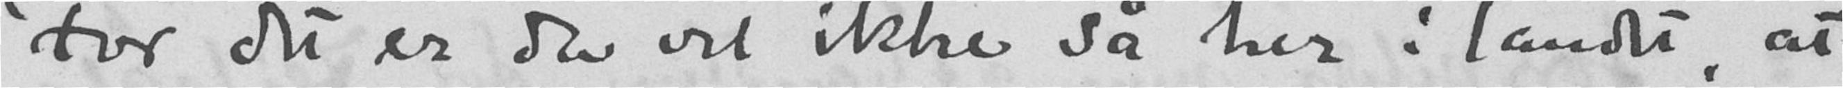For det er da vel ikke så her i landet, at
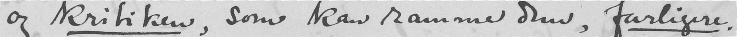og kritiken, som kan ramme dem, farligere.
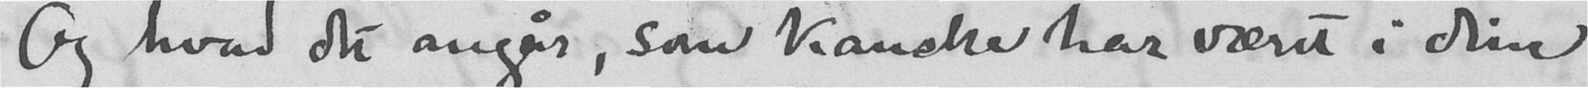Og hvad det angår, som kanske har været i dine
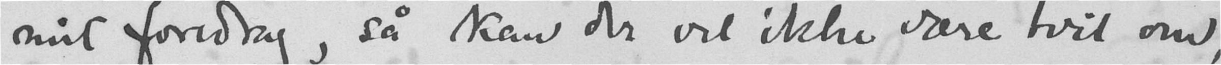mit foredrag, så kan der vel ikke være tvil om,
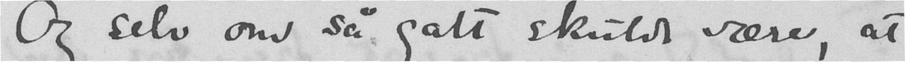Og selv om så galt skulde være, at
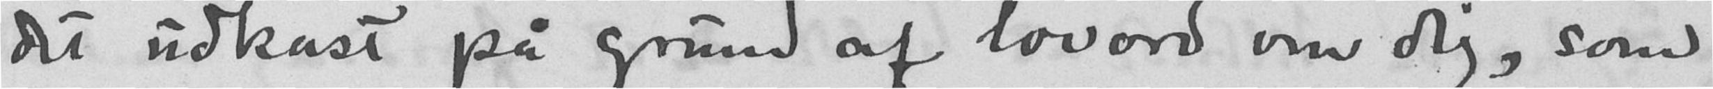det udkast på grund af lovord om dig, som
